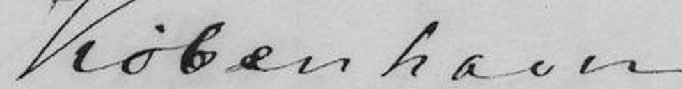København
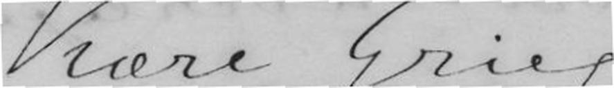Kære Grieg,
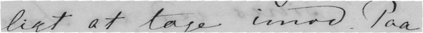ligt at tage imod. Paa
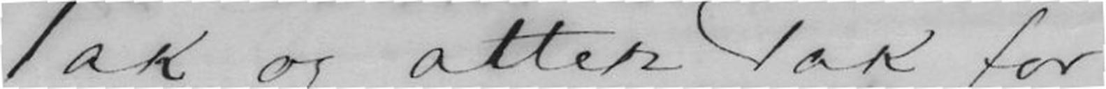Tak og atter Tak for
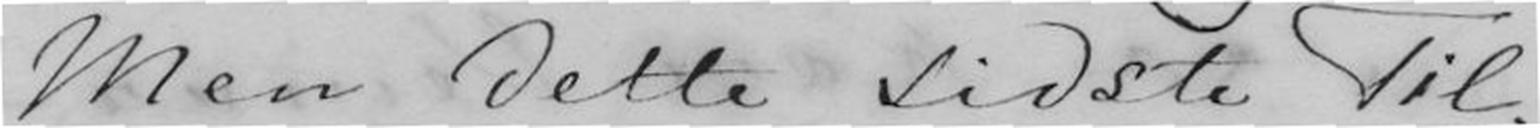Men dette sidste Til-
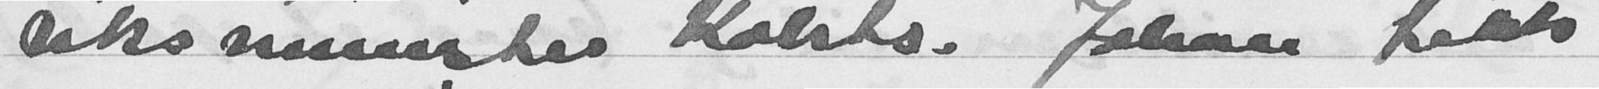riksminister Kohts. Johan fikk
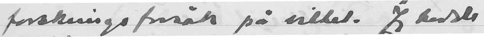forskningsforsøk på viltet. Jeg hadde
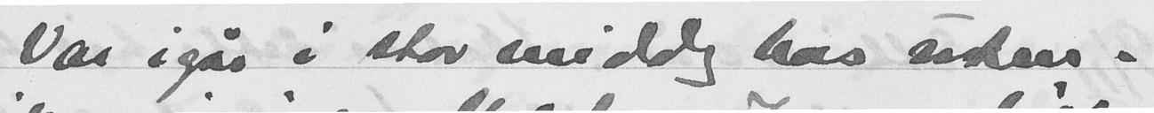Var igår i stor middag hos uten-
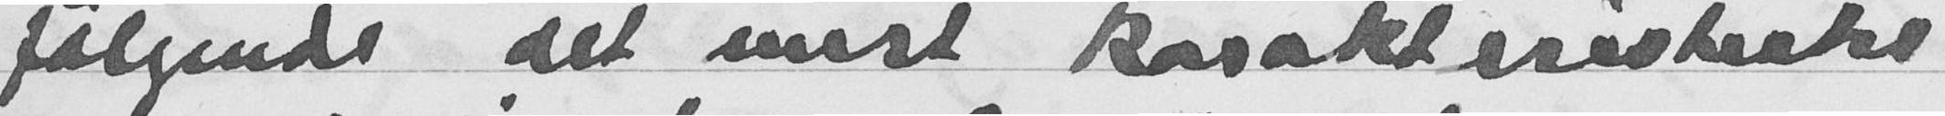følgende det mest karakterestiske
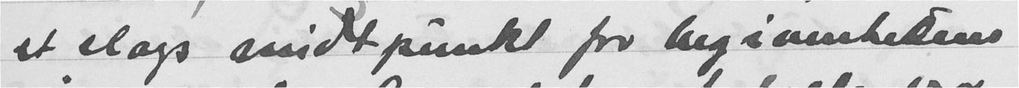et slags midtpunkt for begivenheten
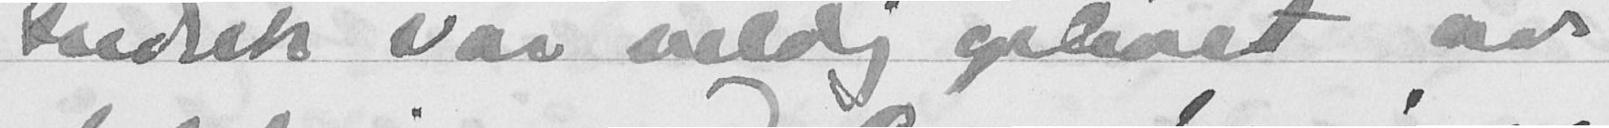Fredrik var veldig oplivet av
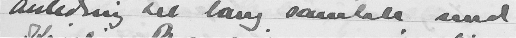anledning til lang samlale med
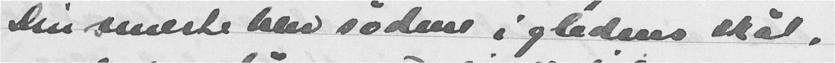Din smerte blev sødme i gledens skål,
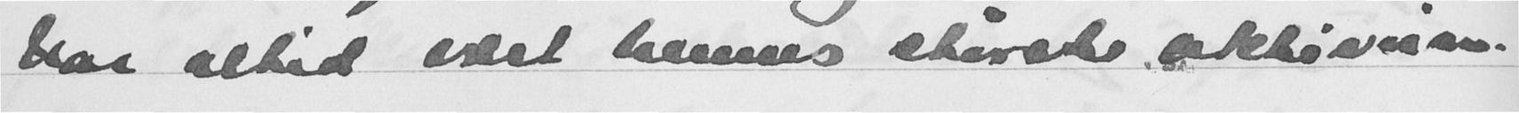har altid vært hennes største aktivier.
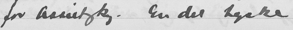for baantzkg. Er det tyske
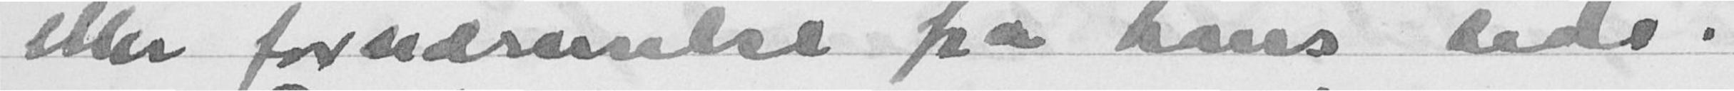eller fornærmelse fra hans side.
